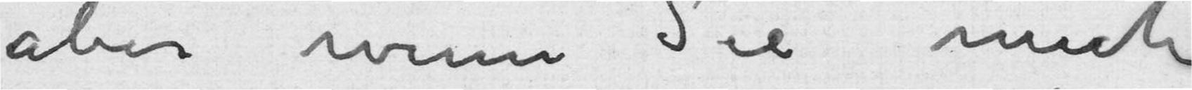aber wenn Sie mich
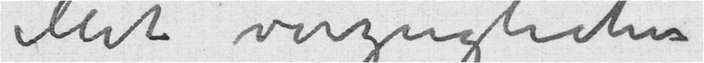Mit vorzuglichen
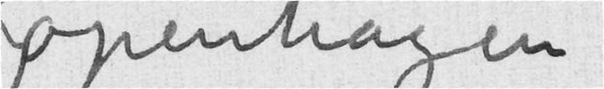Kopenhagen
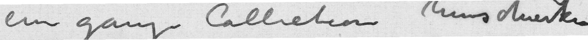ein ganze Collection hinschiecken
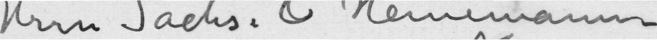Hrrn Sachse & Heinemann
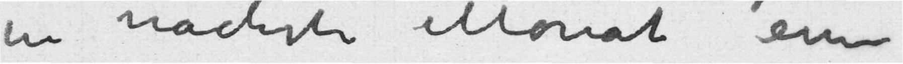in nachste Monat eine
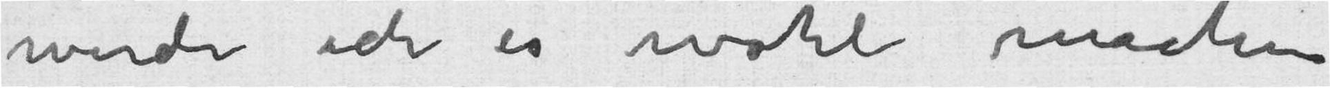werde ich es wohl machen
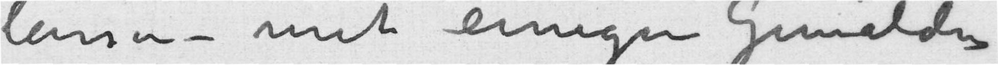lassen – mit einiger Gemalden –
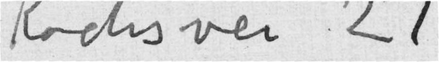Kochsvei 21
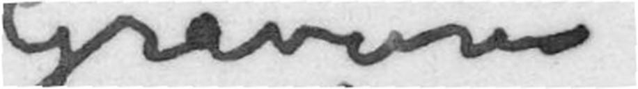Gravuren
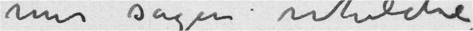mir sagen whelche
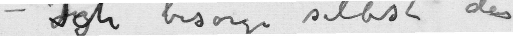– Ich besorge selbst das
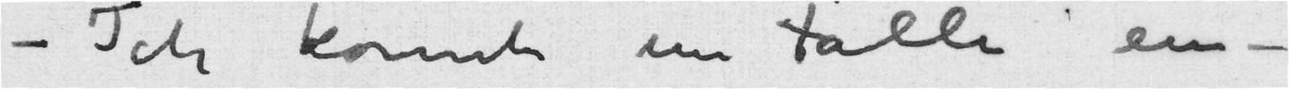– Ich konnte im Falle ein
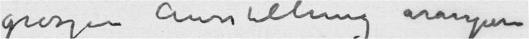groszen Ausstellung arangieren
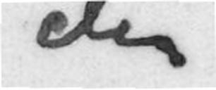der
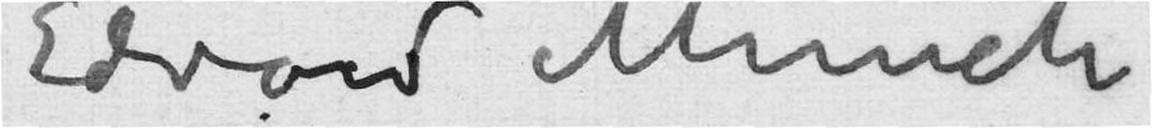Edvard Munch
